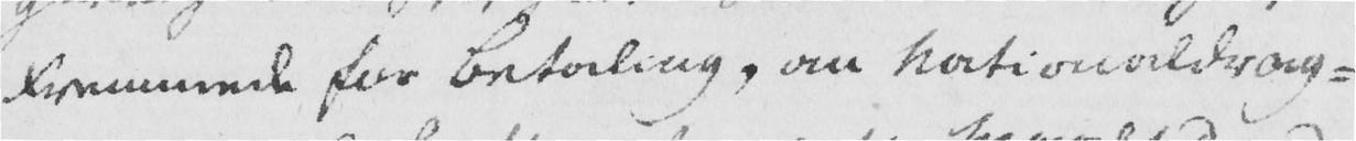Fremmede for Betaling, om Nationaldrag-
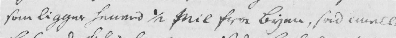som ligger henved ½ mil fra Byen, sad imell.
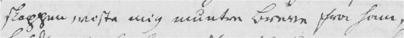skoppen, viste mig muntre Breve fra ham,
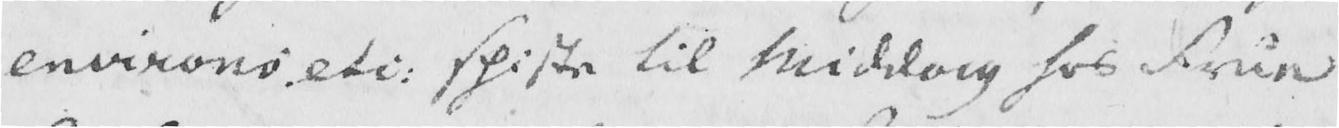environs etc. spiste til Middag hos Frue
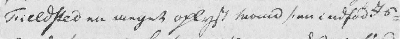Fieldsted en meget oplyst Mand /: en indfød Is-
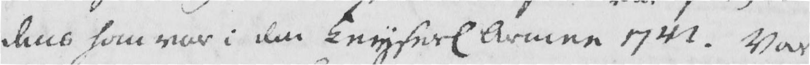dens han var i den Keyserl Armee 1741. Var
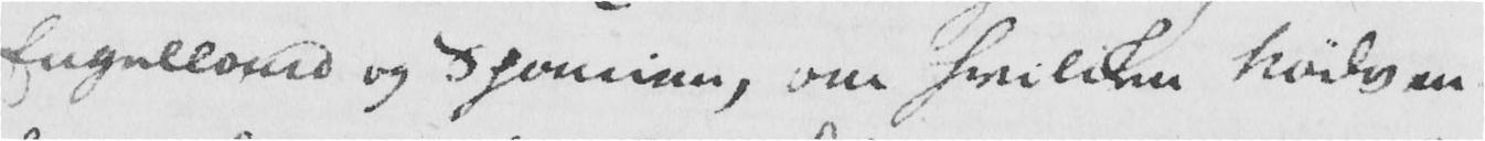Engelland og Spanien, om hvilken Nødven-
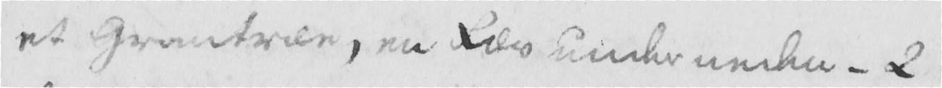et Grantræe, en Ræv under neden - 2
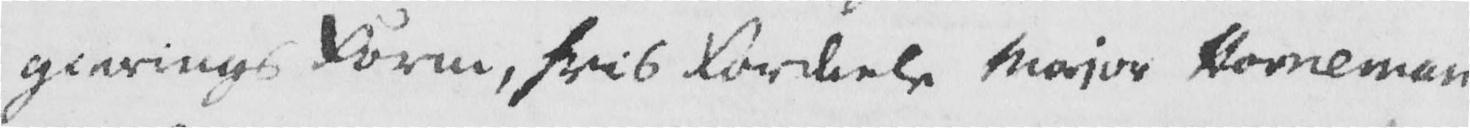gierings Form, hvis Fordeele Major Horneman
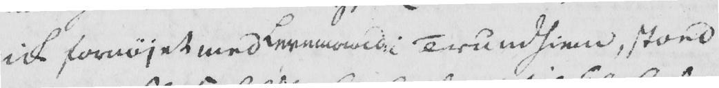icke fornøjet med Levemaad i Trundhiem, stoed
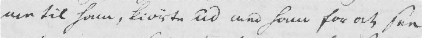me til ham, kiørte ud med ham for at see
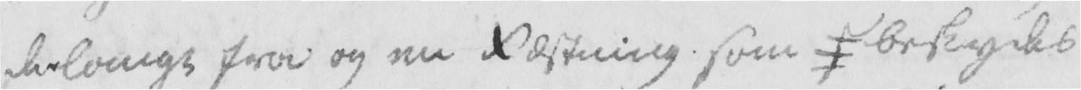der langt fra og en Fæstning som beskydes.
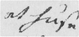at huse
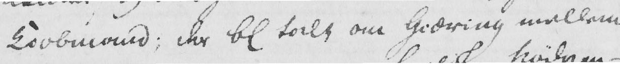Kiøbmand; der bl talt om Giæring mellem
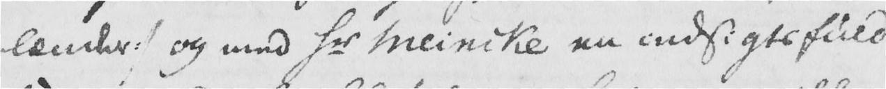lænder :/ og med Hr Meincke en indsigtsfuld
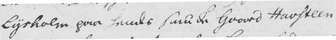Lysholm paa hendes smukke Gaard Havsteen
